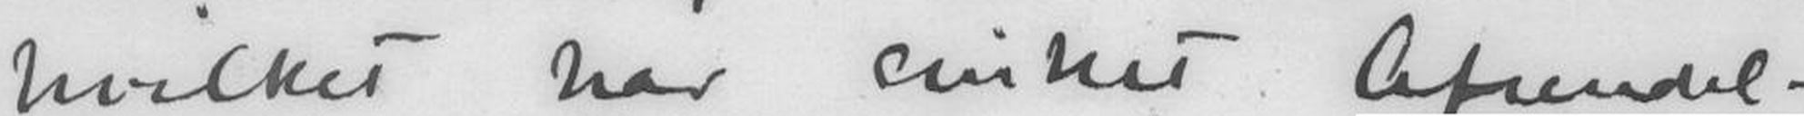hvilket har sinket Afsendel-
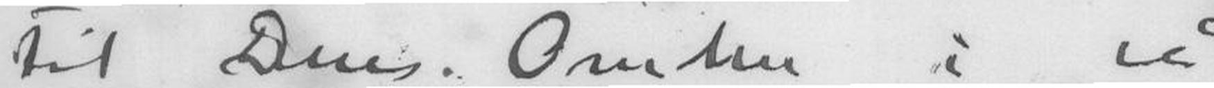til Dem. Om den i så
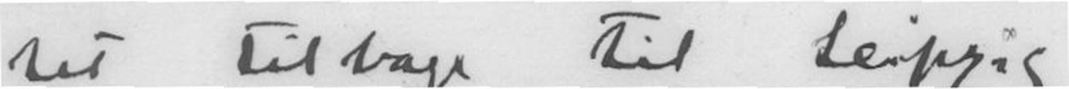tet tilbage til Leipzig
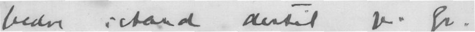bedre istand dertil p. Gr.
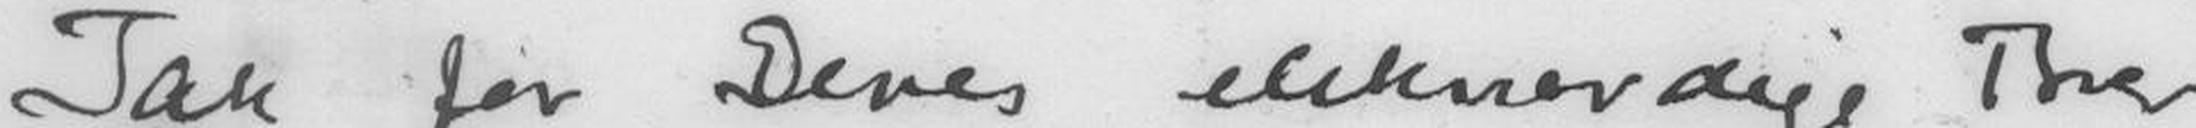Tak for Deres elskværdige Brev
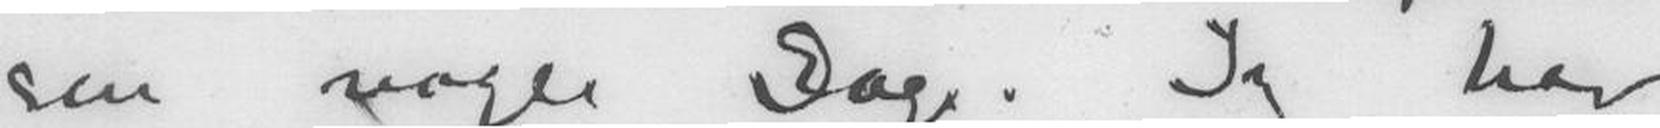sen nogle Dage. Jeg har
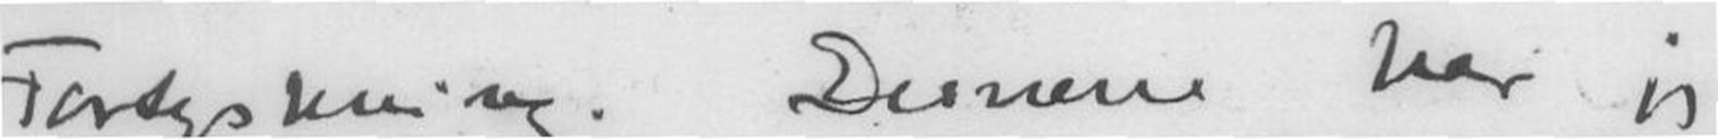Fortyskning. Desværre har jeg
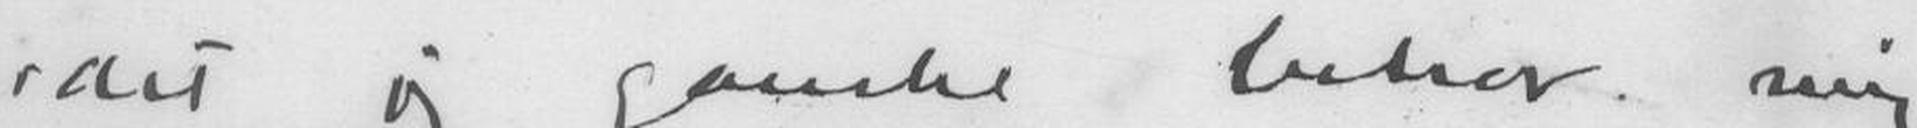idet jeg ganske betror mig
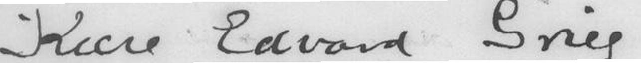Kære Edvard Grieg!
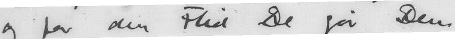og for den Flid De gør Dem
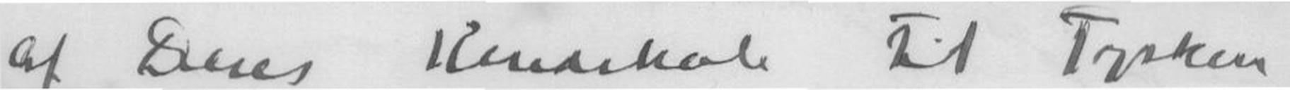af Deres Kendskap til Tysken
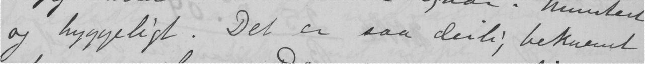og hyggeligt. Det er saa deilig bekvemt
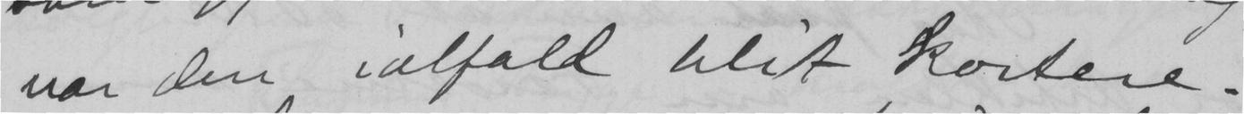var den ialfald blit kortere.
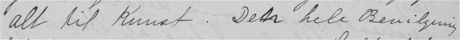alt til Kunst. Den hele Bevilgning
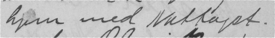hjem med Nattoget.
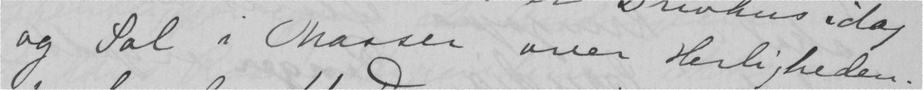og Sol i Masser over Herligheden.
